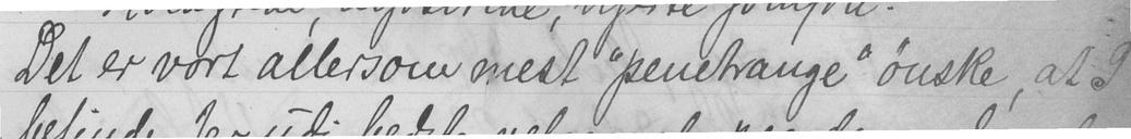Det er vort allersom mest "penetrange" ønske, at I
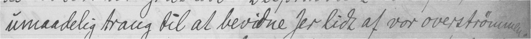umaadelig trang til at bevidne Jer lidt af vor overstrømmen-
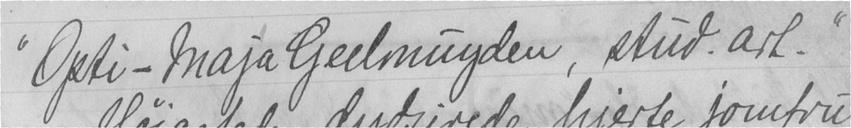"Opti-Maja Geelmuyden, stud. art."
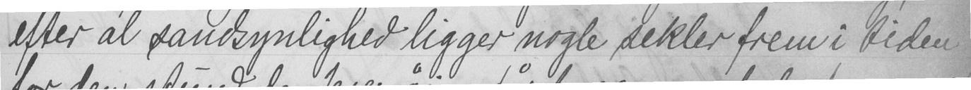efter al sandsylighed ligger nogle sekler frem i tiden
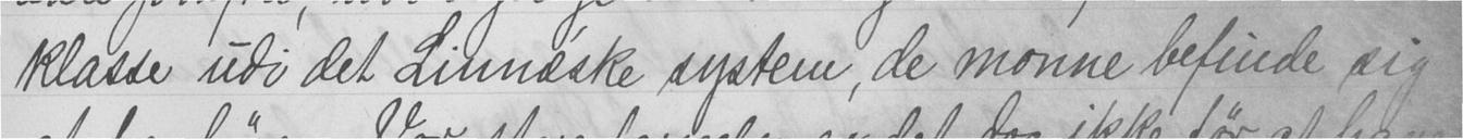klasse udi det Linnæske system, de monne befinde sig
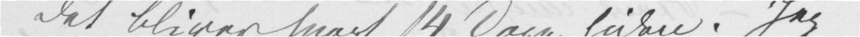Det bliver snart 14 Dage siden. Jeg
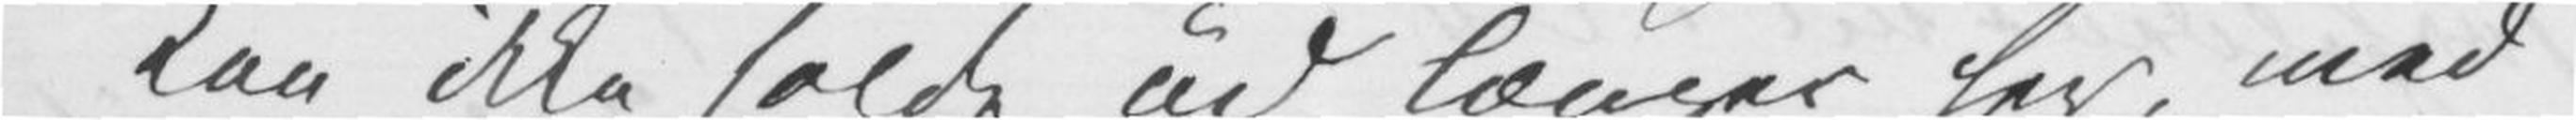kan ikke holde ud længer her, med
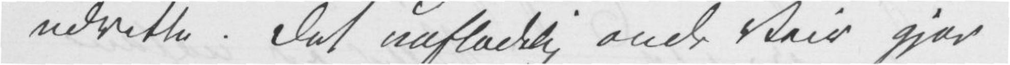udrette. Det uafladelig onde Veir gjør
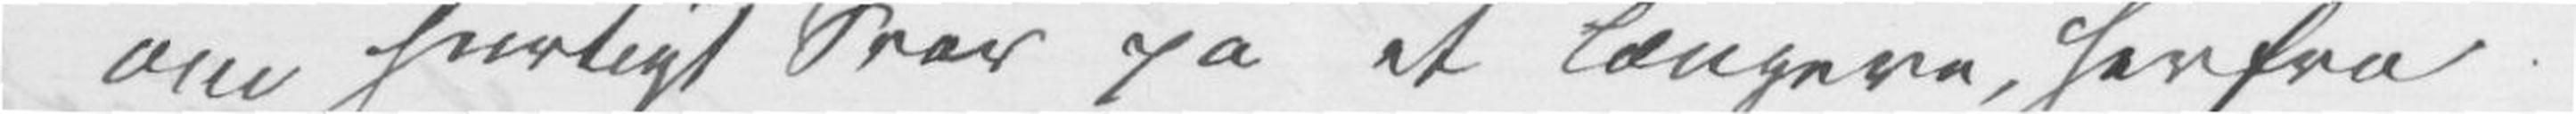om hurtigt Svar på et længere, herfra
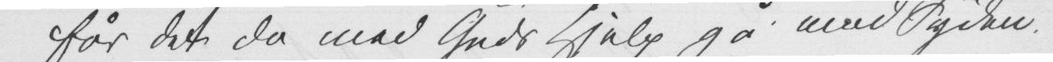får det da med Guds Hjelp gå mod Syden,
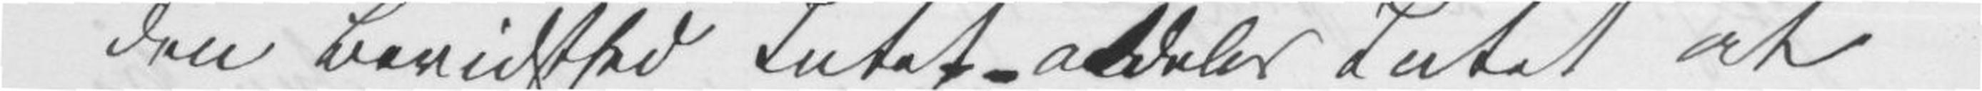den Bevidsthed Intet – aldeles Intet at
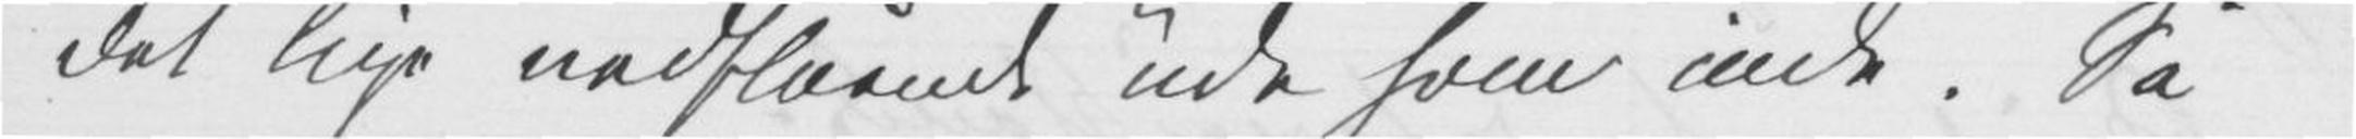det lige nedslående ude som inde. Så
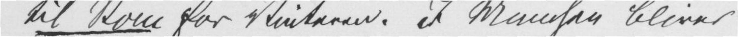til Rom for Vinteren. I München bliver
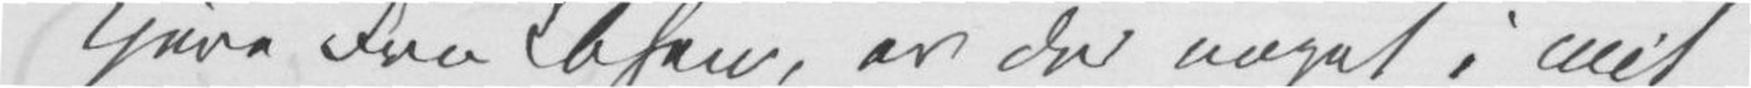Kjere Fru Ibsen, er der noget i mit
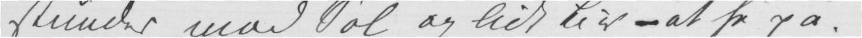stunder mod Sol og lidt Liv – at se på.
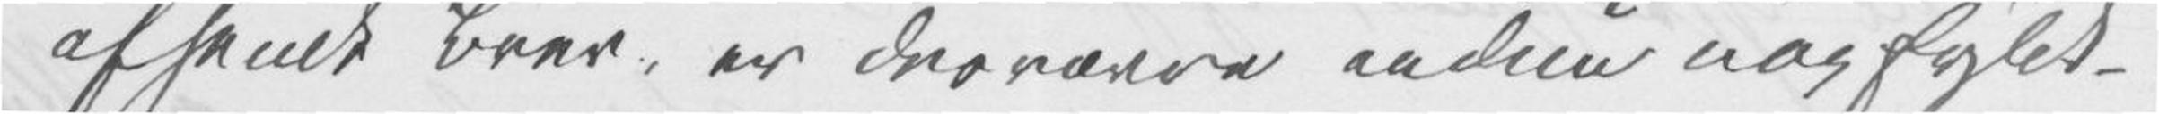afsendt Brev, er desværre endnu uopfyldt.
